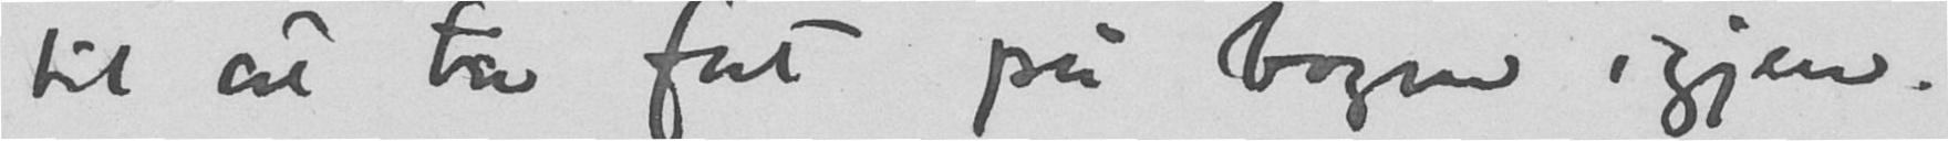til at ta fat på bogen igjen.
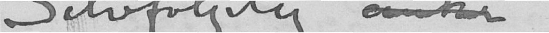Selvfølgelig antar
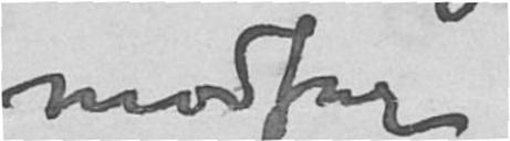modtar
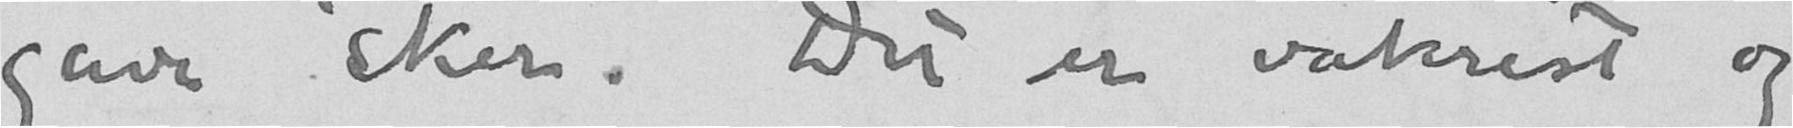gave sker. Det er vakrest og
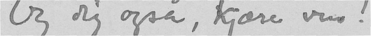Og deg også, kjære ven!
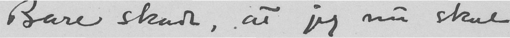Bare skade, at jeg nu skal
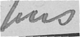Jens
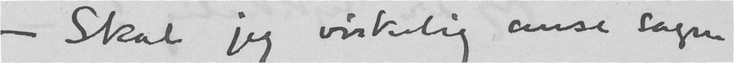- Skal jeg virkelig anse sagen
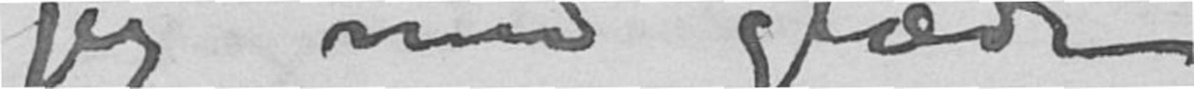jeg med glæde
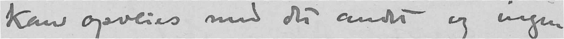kan opveies med det andet og ingen
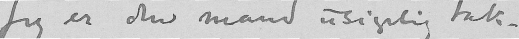Jeg er den mand usigelig tak-
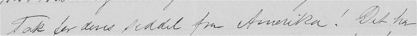Tak for deres seddel fra Amerika! Det har
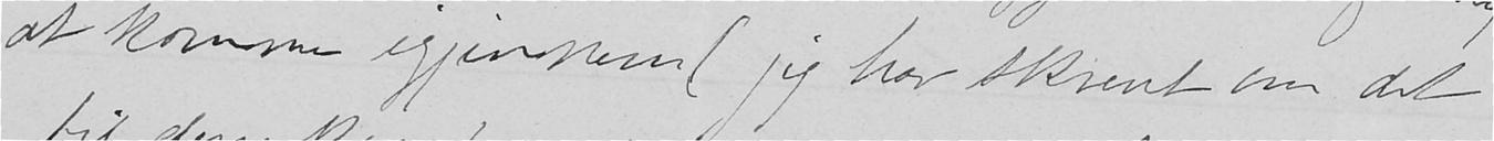at komme igjennem (jeg har skrevet em det
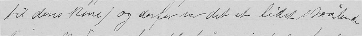til deres kone) og derfor var det et lidet strålende
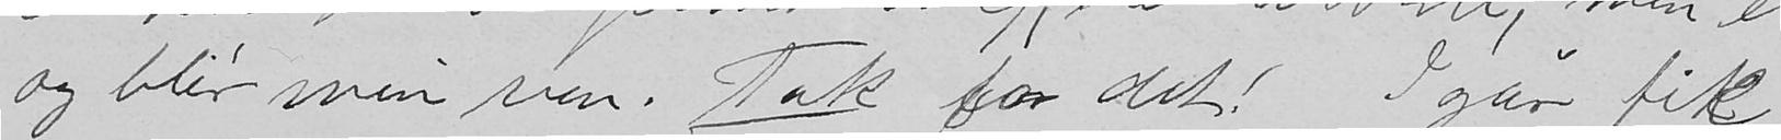og blir min ven. Tak for det! Igår fik
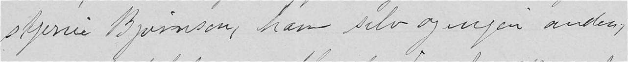stjerne Bjørnson, han selv og ingen andre,
